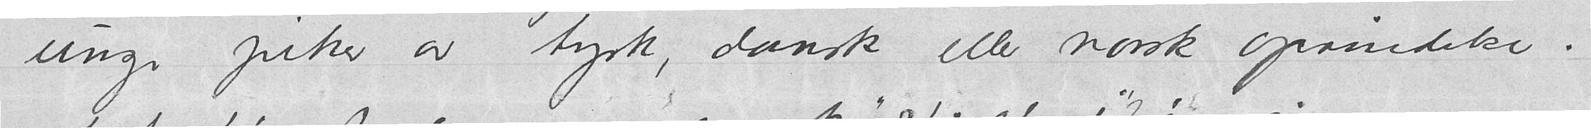unge piker av tysk, dansk eller norsk oprindelse.
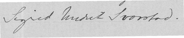Sigrid Undset Svarstad.
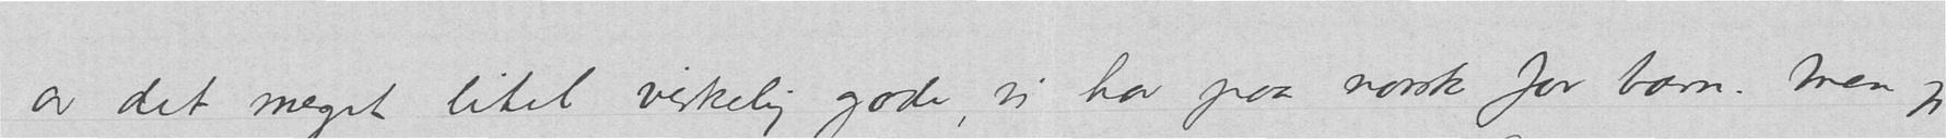av det meget litet virkelig gode vi har paa norsk for barn. Men jeg
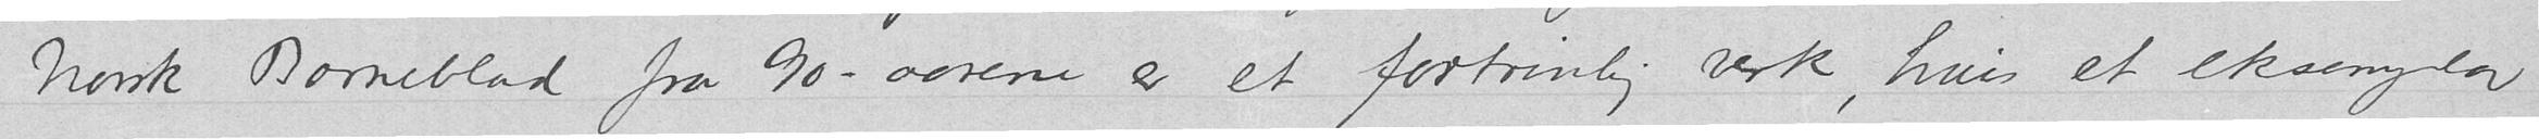Norsk Barneblad fra 90- aarene er et fortrinlig verk, hvis et eksemplar
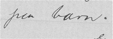paa barn.
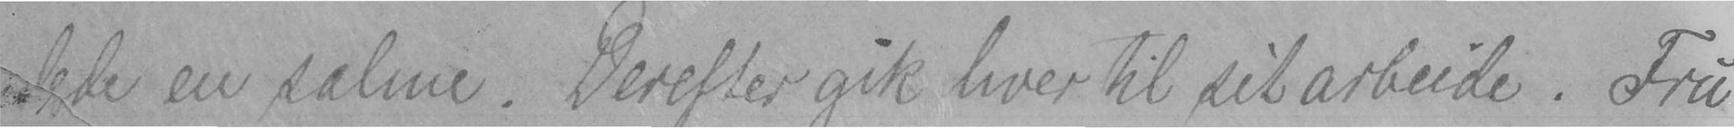lede en salme. Derefter gik hver til sit arbeide. Fru
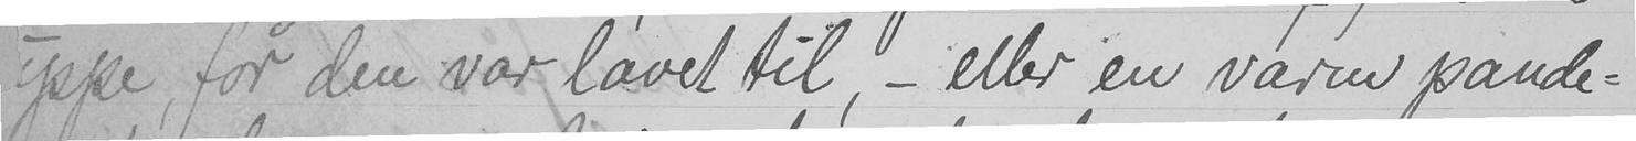suppe, for den var lavet til, - eller en varm pande-
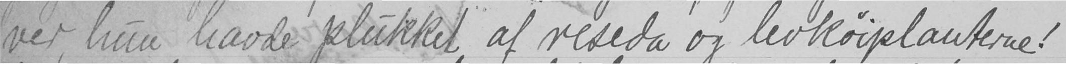ver, hun havde plukket af reseda og levkøiplanterne!
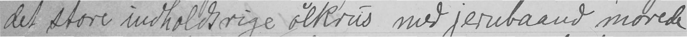det store indholdsrige ølkrus med jernbaand morede
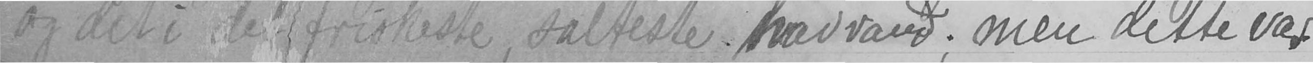og det i det friskeste, salteste havvand; men dette var
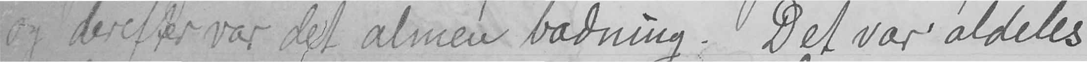og derefter var det almen badning. Det var aldeles
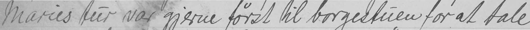Maries tur var gjerne først til borgestuen for at tale
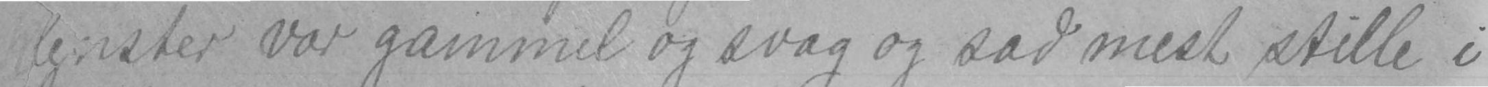Mynster var gammel og svag og sad mest stille i
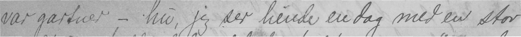var gartner - hu, jeg ser hende en dag med en stor
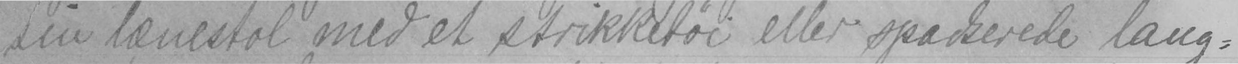sin lænestol med et strikketøi eller spadserede lang-
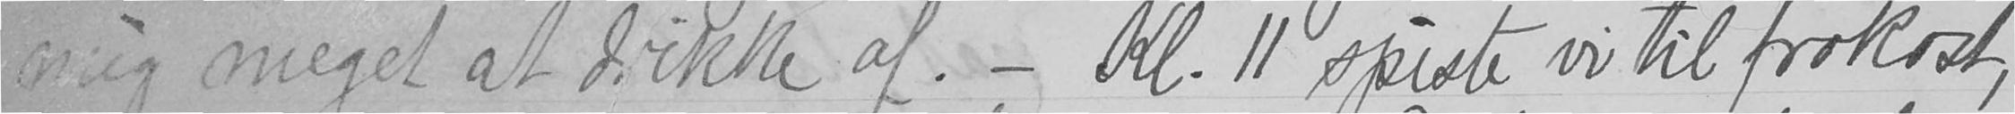mig meget at drikke af. - Kl. 11 spiste vi til frokost,
